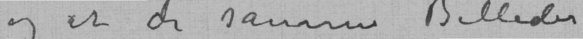og at de samme Billeder
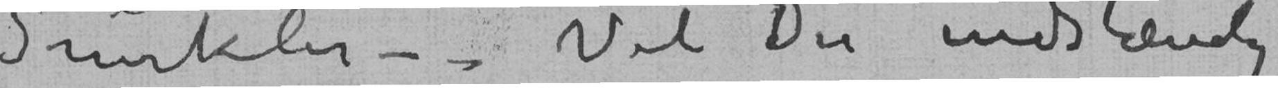Snirkler – Vil Du indstændig
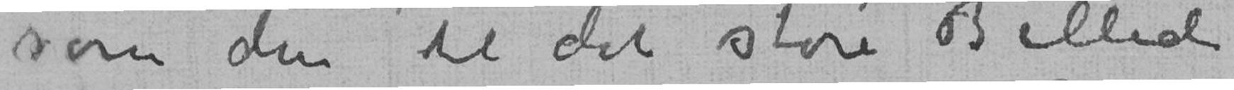som den til det store Billede
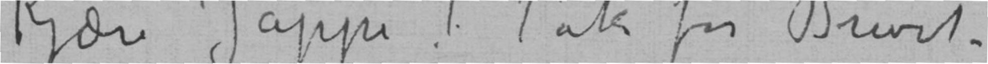Kjære Jappe! Tak for Brevet –
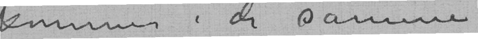kommer i de samme
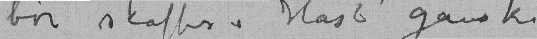bør skaffes i Hast ganske
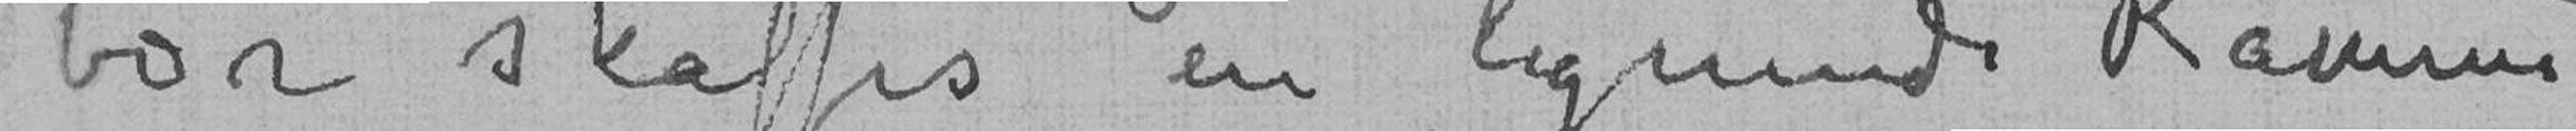bør skaffes en lignende Ramme
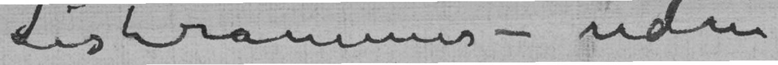Listrammer – uden
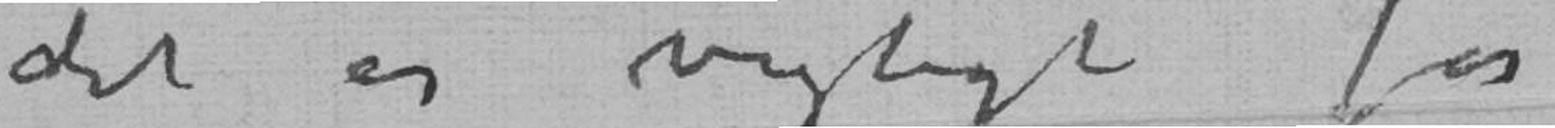det er vigtigt for
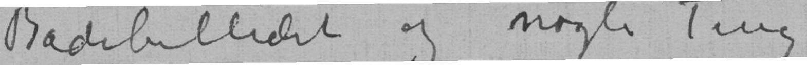Badebilledet og nogle Ting
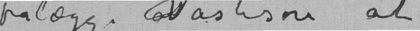pålægge Vasteson at
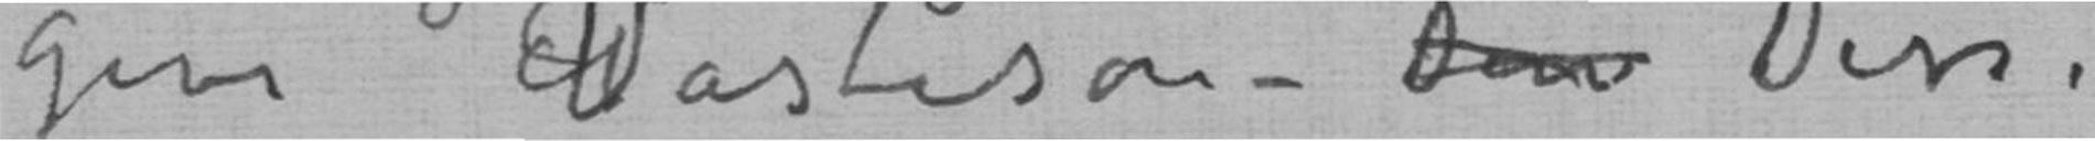give Vasteson – D Disse
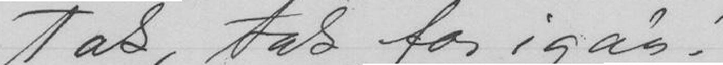Tak, tak for igår!
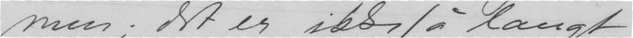men; det er ikke så langt
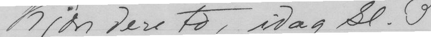Kjære dere to, idag kl. 3
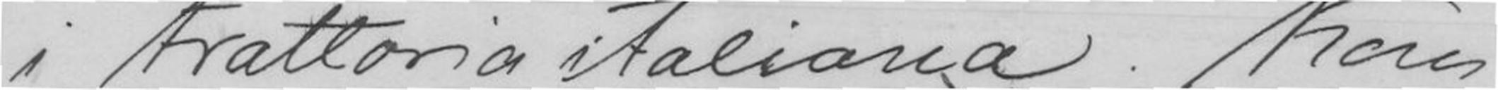i Trattoria italiana. Kom
